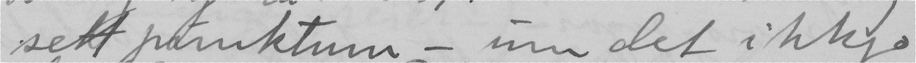sett punktum – um det ikkje
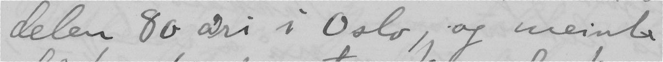delen 80 åri i Oslo, og meinte
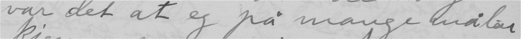var det at eg på mange måtar
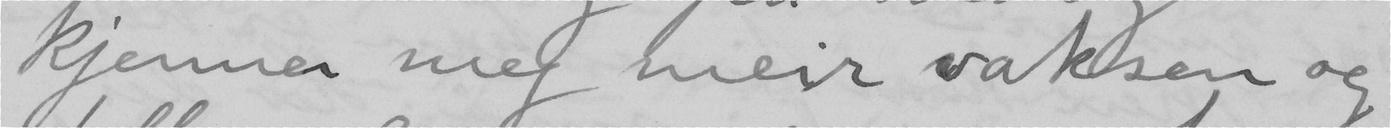kjenner meg meir vaksen og
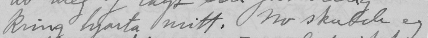kring hjarta mitt. No skulde eg
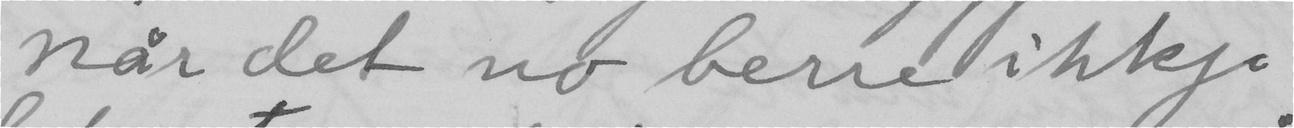Når det no berre ikkje
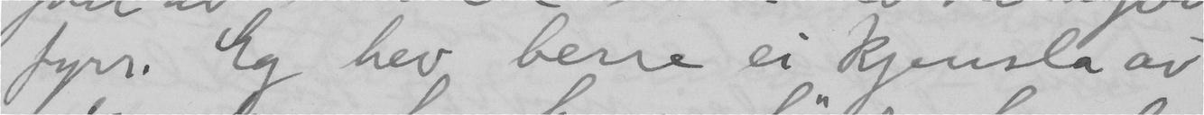fyrr. Eg hev berre ei kjensla av
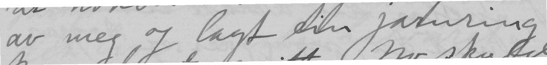av meg og lagt ein jarnring
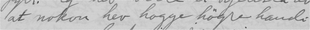at nokon hev hogge högre handi
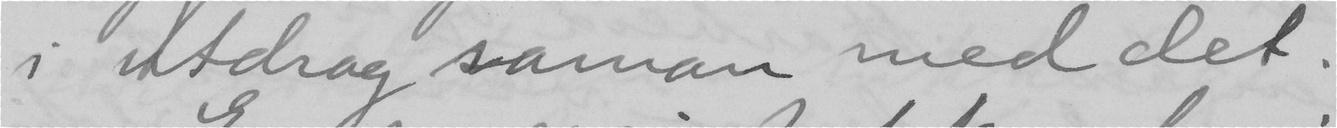i utdrag saman med det.
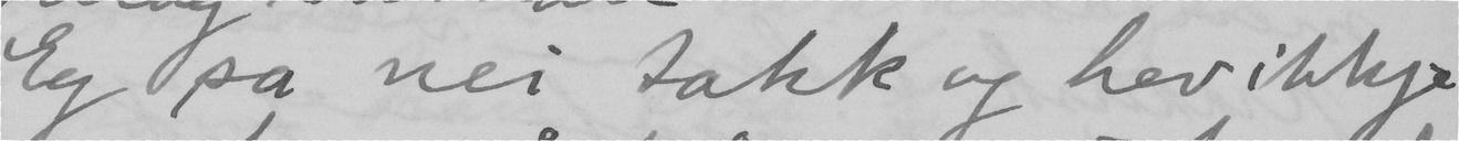Eg sa nei takk og hev ikkje
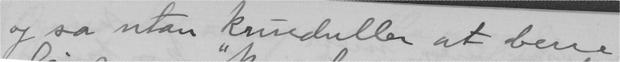og sa utan krusduller at berre
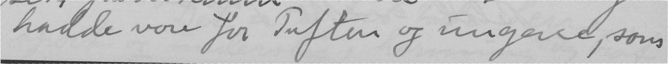hadde vore for Tuften og ungane, som
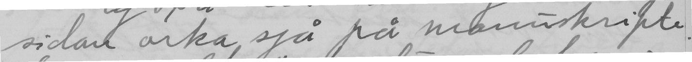sidan orka sjå på manuskripte.
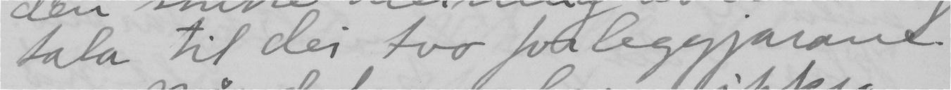tala til dei tvo forleggjarane.
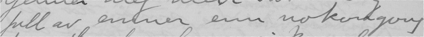full av enmner enn nokon gong
